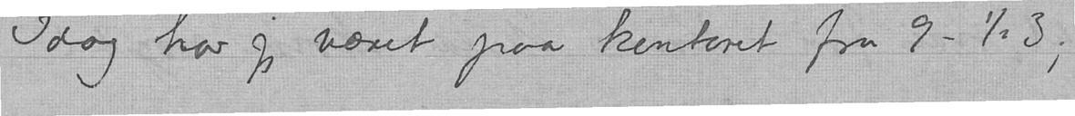Idag har jeg været paa kontoret fra 9 - ½ 3,
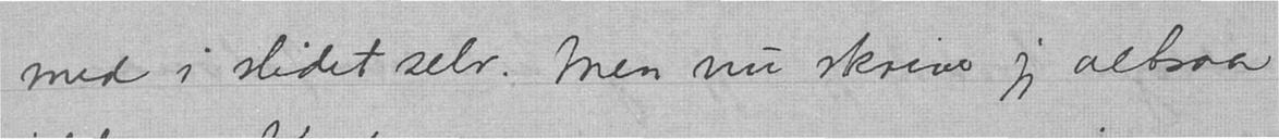med i slidet selv. Men nu skriver jeg altsaa
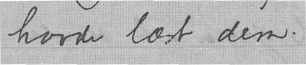havde læst dem.
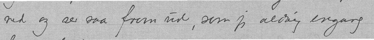ned og ser saa from ud, som jeg aldrig engang
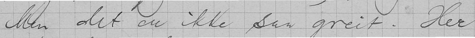Men det er ikke saa greit. Her
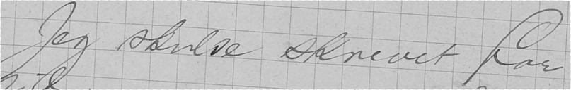Jeg skulde skrevet for
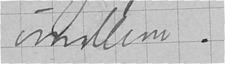imellem.
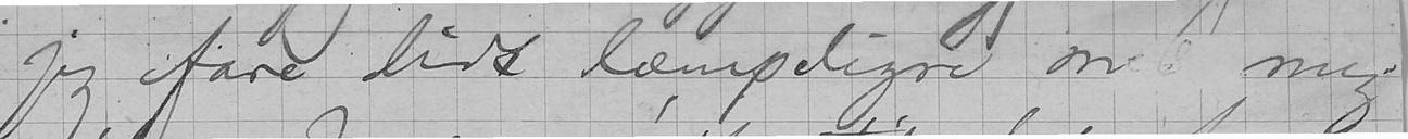jeg fare lidt læmpeligre med mig
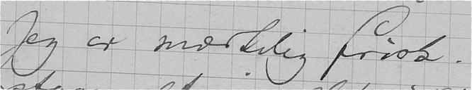Jeg er mærkelig frisk.
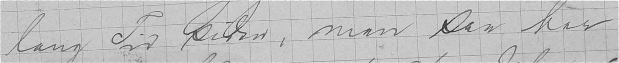lang Tid siden, men saa har
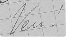Ven!
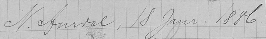N. Aurdal, 18. Janr. 1886.
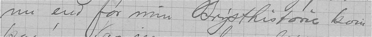nu end før min Brysthistorie kom
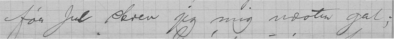før Jul skrev jeg mig næsten gal;
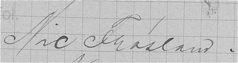Nic Frøsland.
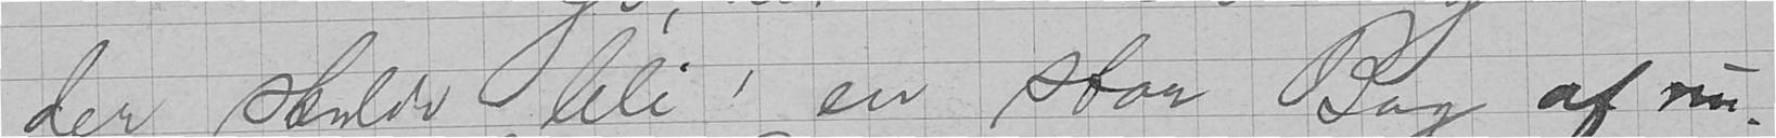der skulde bli en stor Bog af nu.
